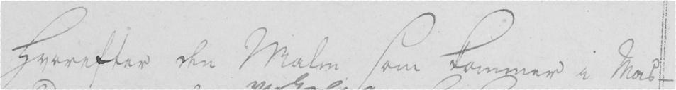Hvorefter den Malm som kommer i Mas-
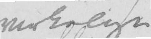virkelige
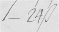1 - 24 s
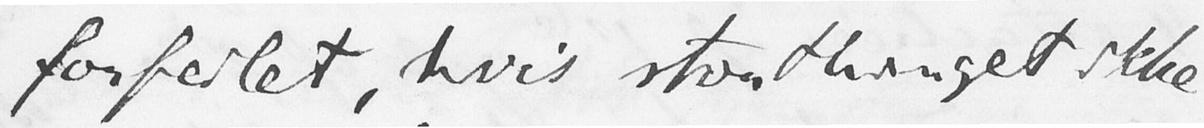forfeilet, hvis storthinget ikke
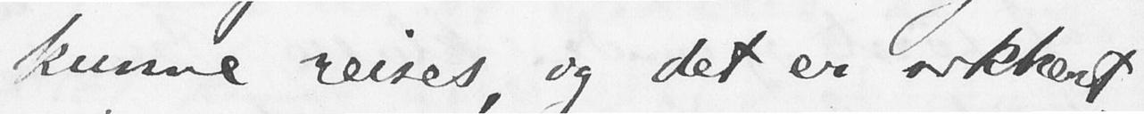kunne reises, og det er sikkert,
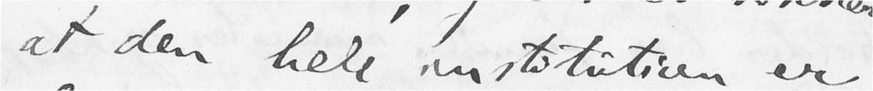at den hele institution er
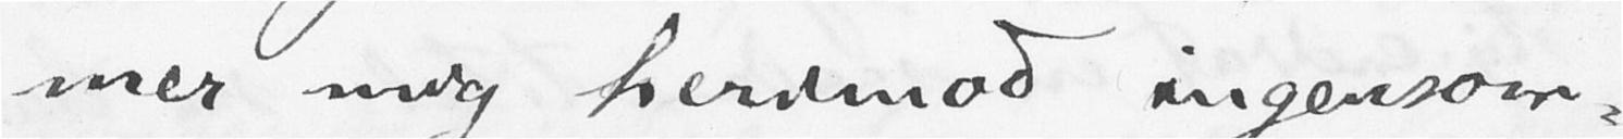mer mig herimod ingensom-
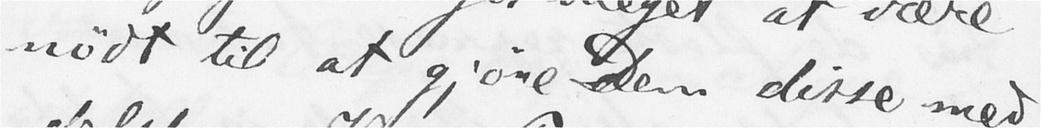nødt til at gjøre Dem disse med-
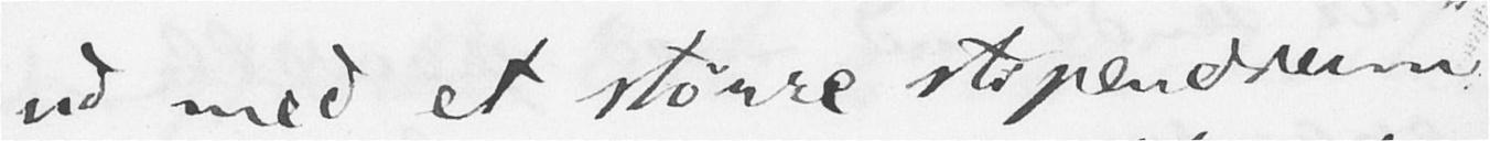ud med et større stipendium
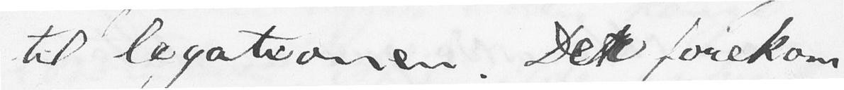til legationen. Det forekom-
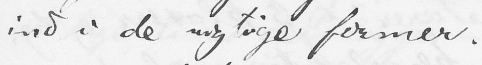ind i de rigtige former.
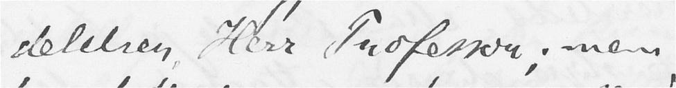delelser, Herr Professor; men
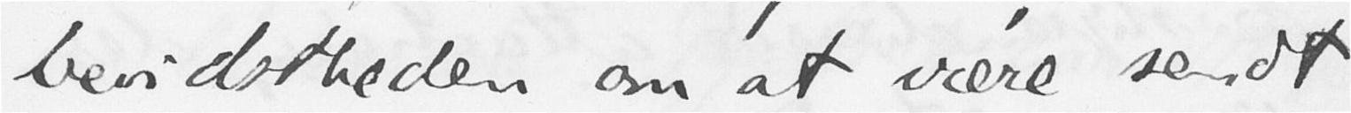bevidstheden om at være sendt
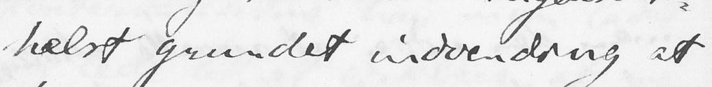helst grundet indvending at
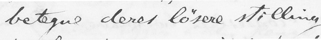betegne deres løsere stilling
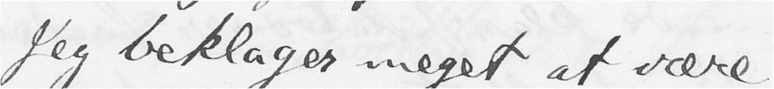Jeg beklager meget at være
